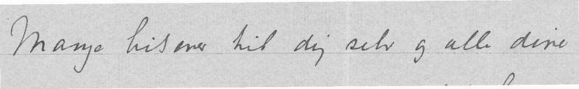Mange hilsener til dig selv og alle dine
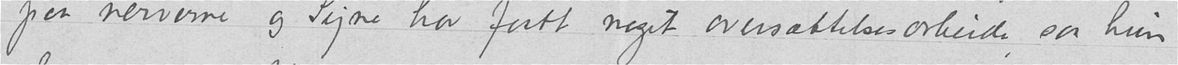paa nerverne og Signe har faatt noget oversættelsesarbeide, saa hun
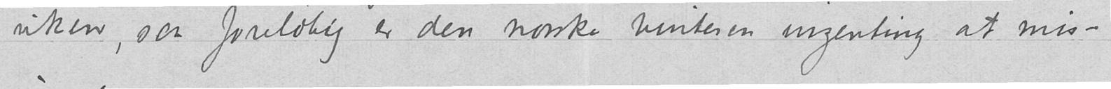uken, saa foreløbig er den norske vinteren ingenting at mis-
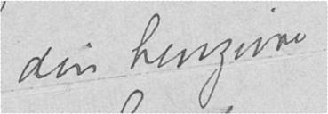din hengivne
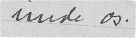unde os.
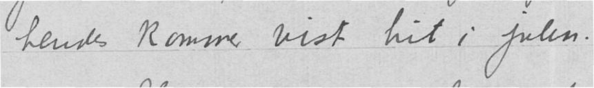hendes kommer vist hit i julen.
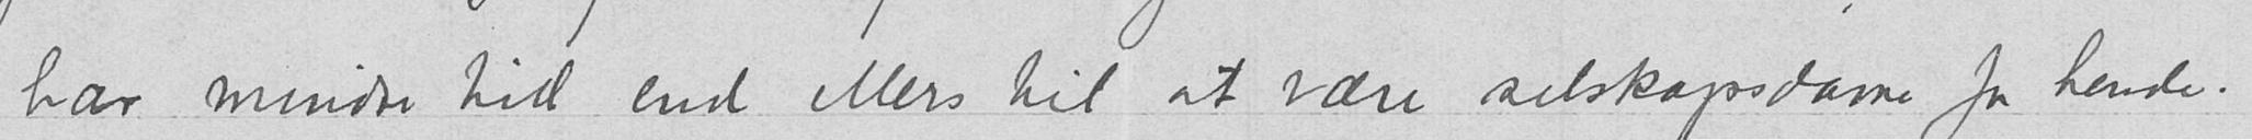har mindre tid end ellers til at være selskapsdame for hende.
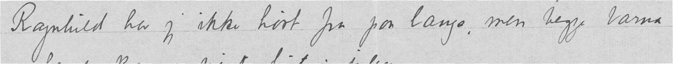Ragnhild har jeg ikke hørt fra paa længe, men begge barna
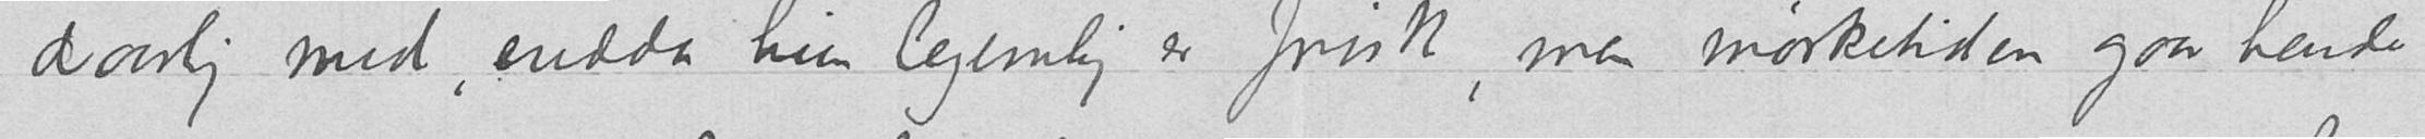daarlig med, endda hun legemlig er frisk, men mørketiden gaar hende
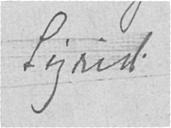Sigrid.
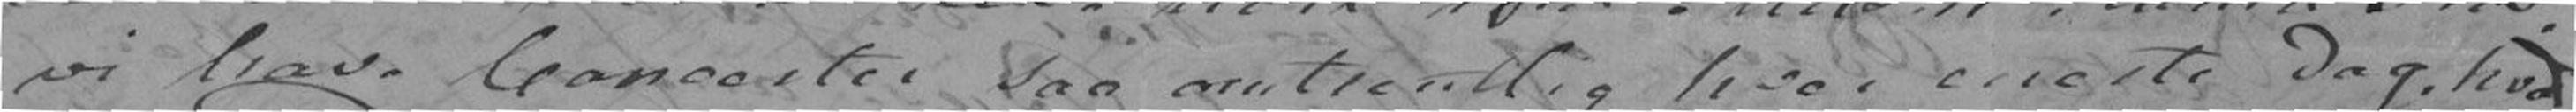vi have Concerter saa omtrentlig hver eneste Dag, hvad
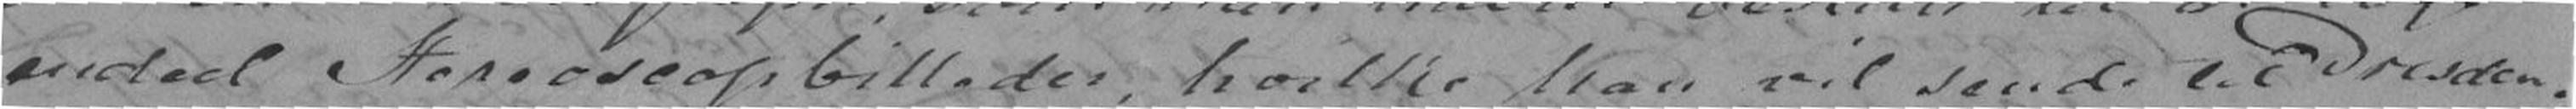endeel Stereoscopbilleder, hvilke han vil sende til Dresden.
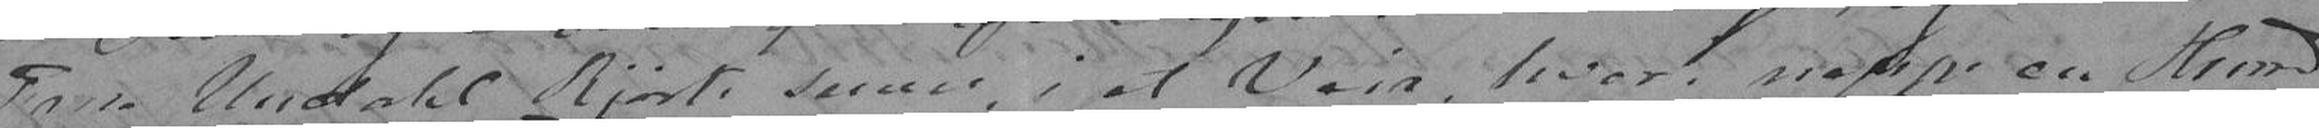Frue Undahl kjørte senere i et Veir, hvor neppe en Hund
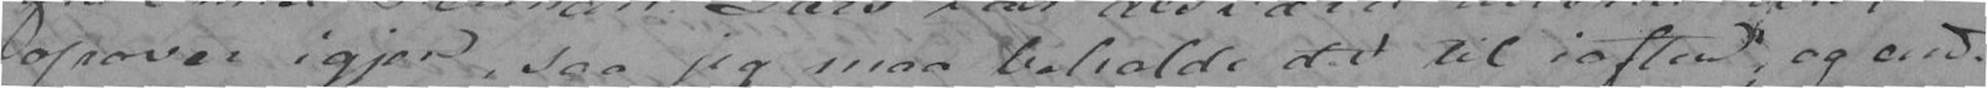opover igjen, saa jeg maa beholde det til iaften, og end-
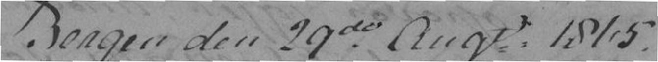Bergen den 29de Augt. 1865.
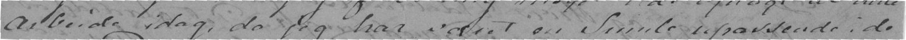Arbeide idag, da jeg har været en Smule upassende i de
